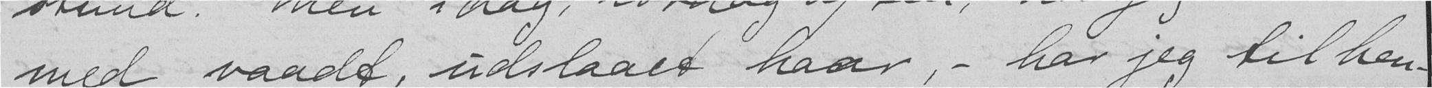med vaadt, udslaaet haar, – har jeg til hen-
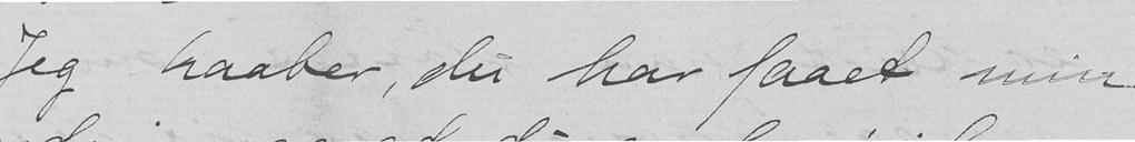Jeg haaber, du har faaet min
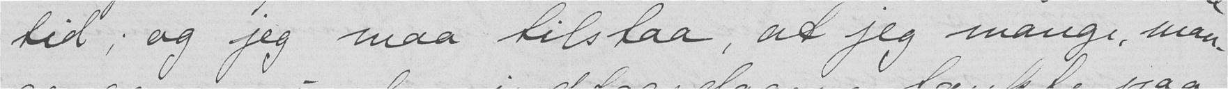tid; og jeg maa tilstaa, at jeg mange, man-
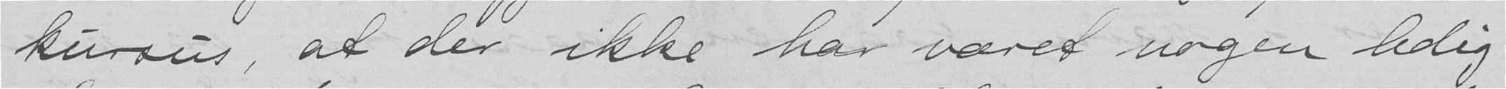kursus, at der ikke har været nogen ledig
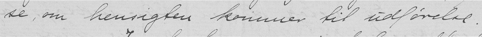se, om hensigten kommer til udførelse.
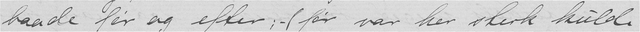baade før og efter; – (før var her sterk kulde
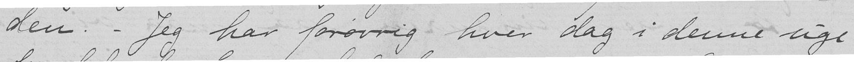den. – Jeg har forøvrig hver dag i denne uge
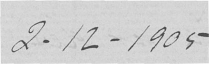2-12-1905
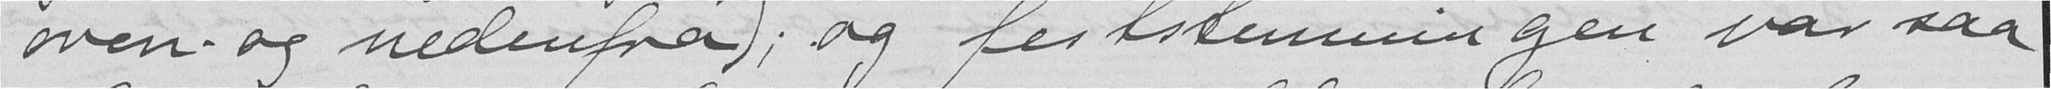oven- og nedenfra); og feststemningen var saa
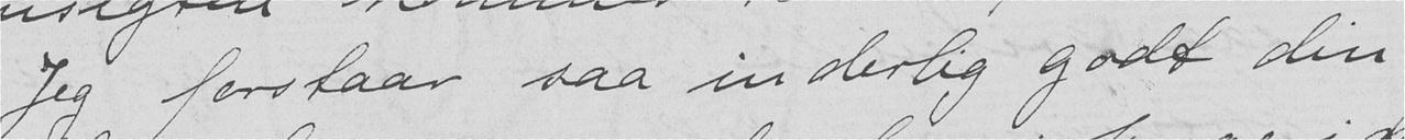Jeg forstaar saa inderlig godt din
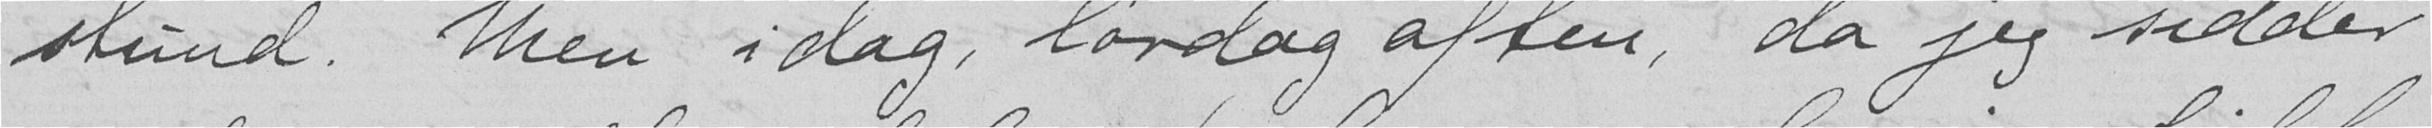stund. Men i dag, lørdag aften, da jeg sidder
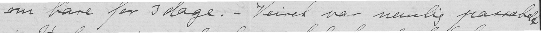om bare for 3 dage. – Veiret var nemlig passabelt
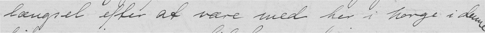længsel efter at være med her i Norge i denne
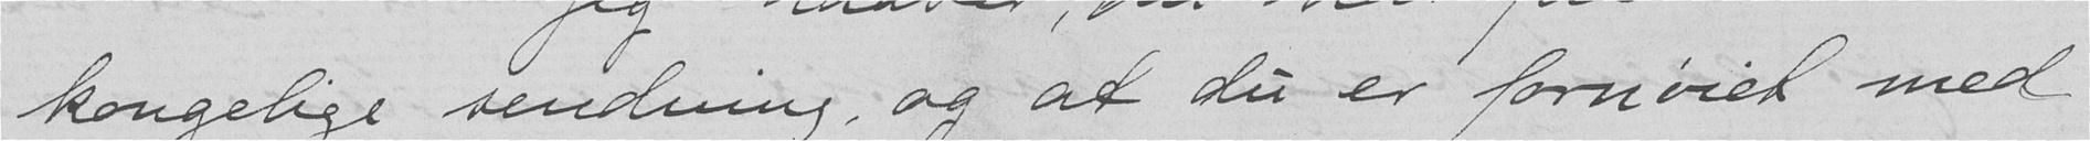kongelige sendning, og at du er fornøiet med
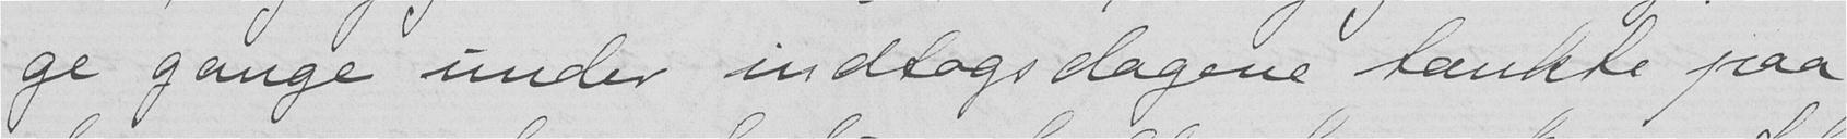ge gange under indtogsdagene tænkte paa
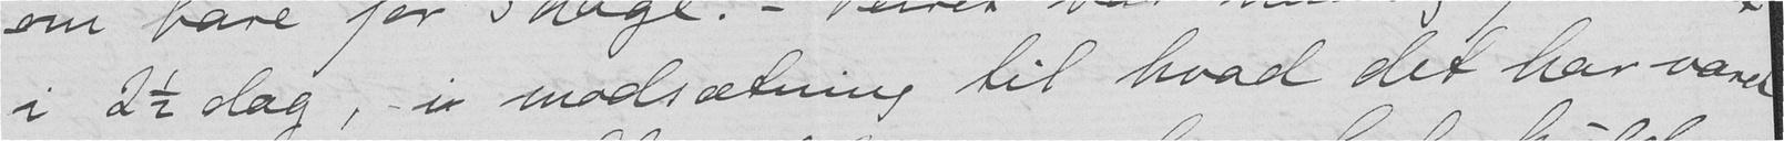i 2 1/2 dag, – i modsætning til hvad det har været
